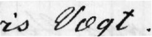vis Vægt.
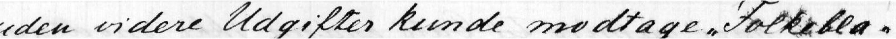uden videre Udgifter kunde modtage Folkebla-
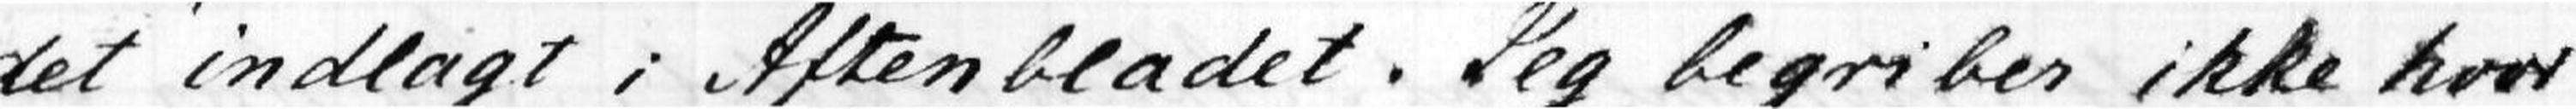det indlagt i Aftenbladet. Jeg begriber ikke hvor-
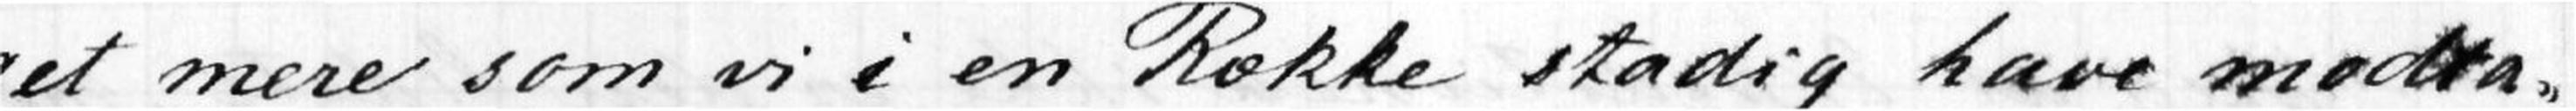get mere som vi i en Række stadig have modta-
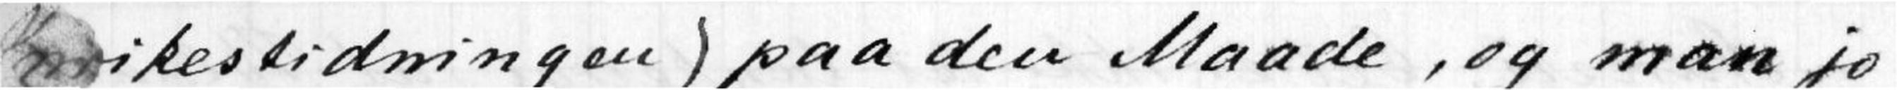rikestidningen) paa den Maade, og man jo
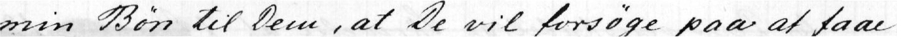min Bøn til Dem, at De vil forsøge paa at faae
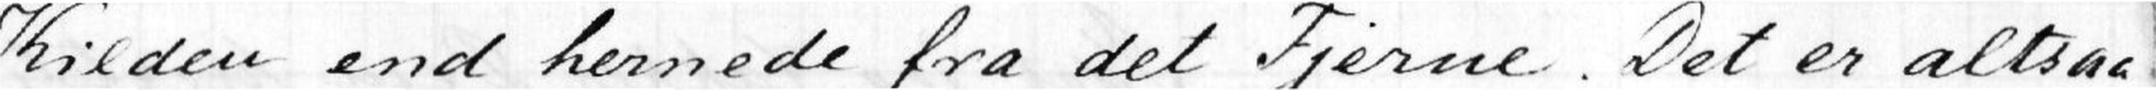Kilden end hernede fra det Fjerne. Det er altsaa
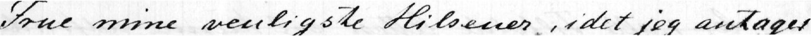Frue mine venligste Hilsener, idet jeg antages
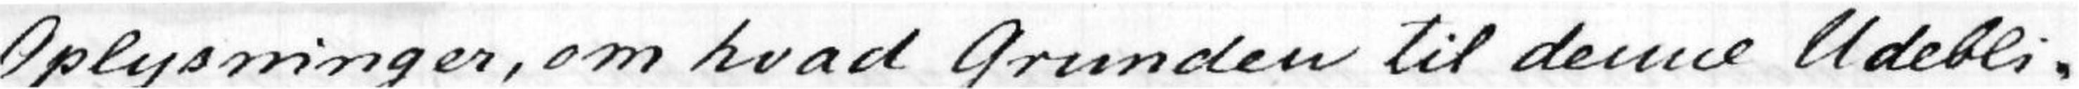Oplysninger, om hvad Grunden til denne Udebli-
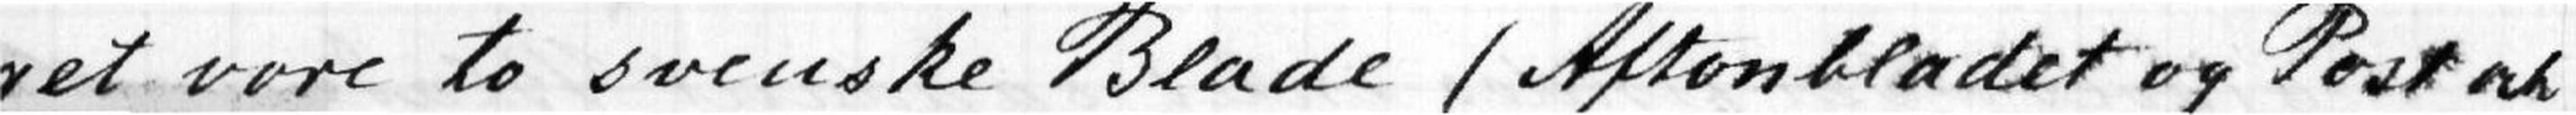get vore to svenske Blade (Aftonbladet og Post och
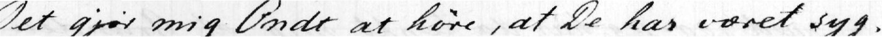Det gjør mig Ondt at høre, at De har været syg,
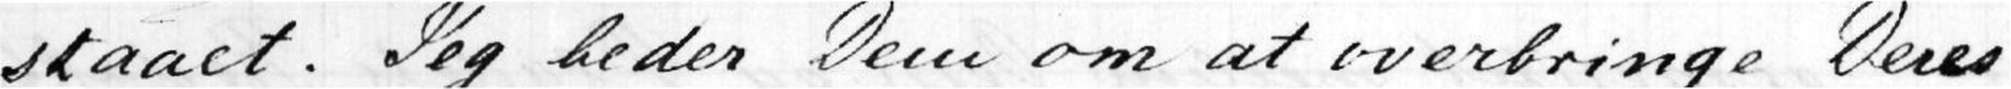staaet. Jeg beder Dem om at overbringe Deres
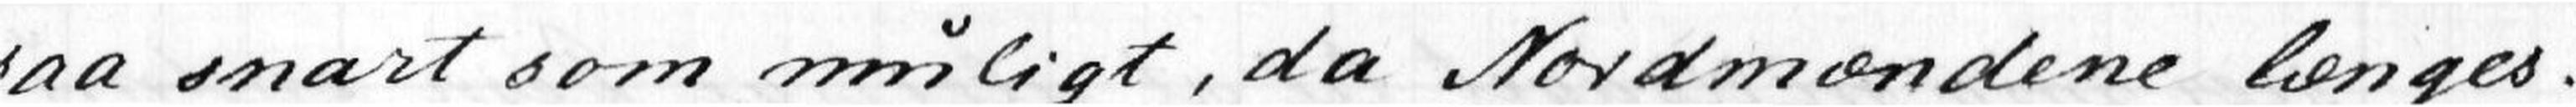saa snart som muligt, da Nordmændene længes
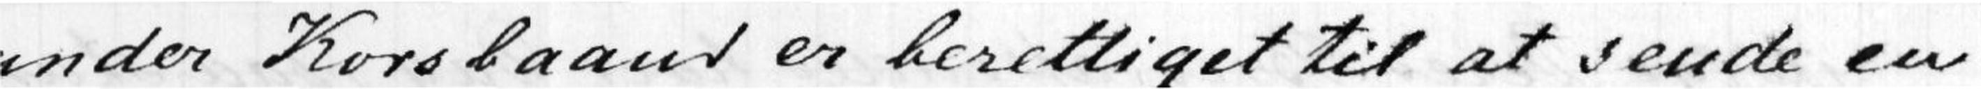under Korsbaand er berettiget til at sende en
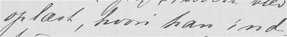oplæst, hvori han ind-
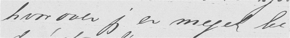hvorover jeg er meget be-
